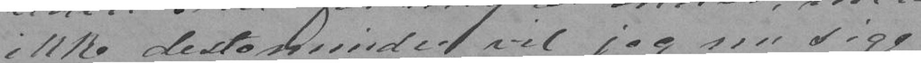ikke destomindre vil jeg nu sige
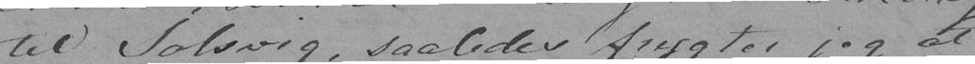til Solsvig, saaledes frygter jeg at
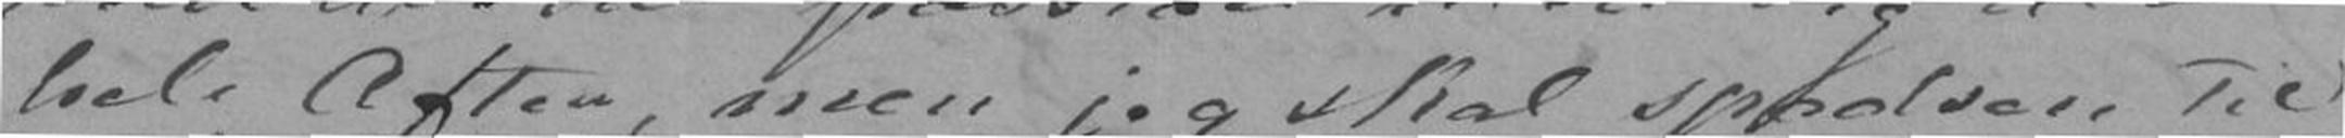hele Aften, men jeg skal spadsere til
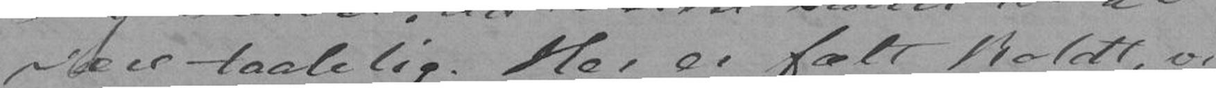være taalelig. Her er fælt koldt, vi
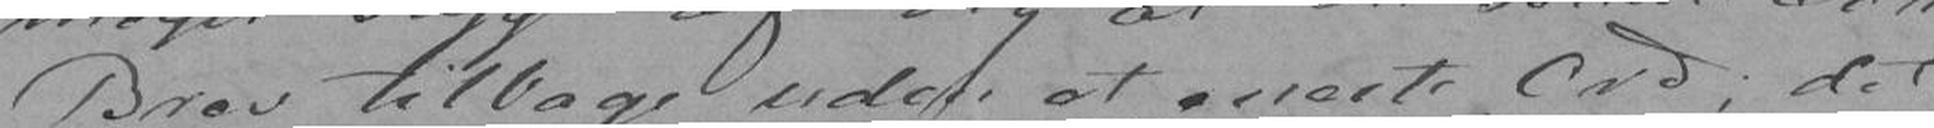Brev tilbage uden et eneste Ord; det
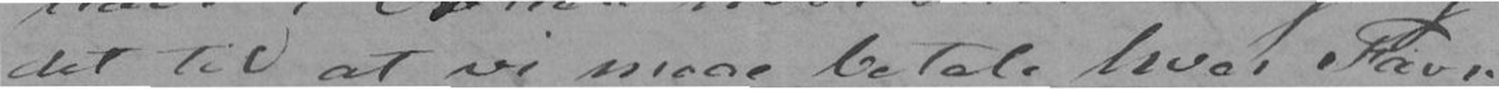det til at vi maae betale hver Favn
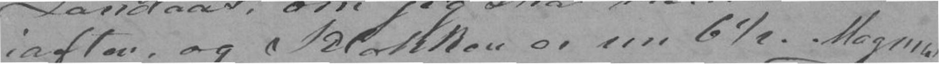iaften, og Klokken er nu 6½. Magnus
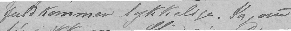fuldkommen lykkelige. Ja, nu
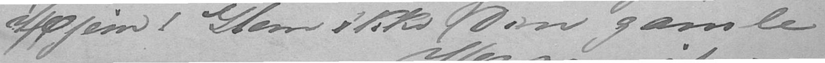Hjem! Glem ikke Din gamle
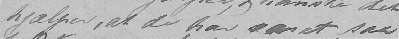hjælper, at de har været saa
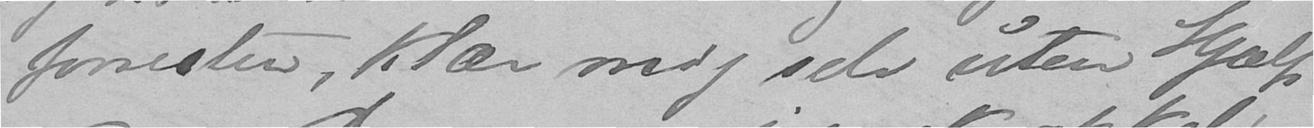forresten, klær mig selv uten Hjælp
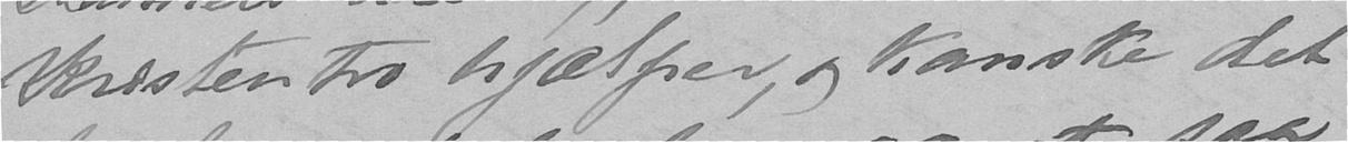Kristen tro hjælper, og kanske det
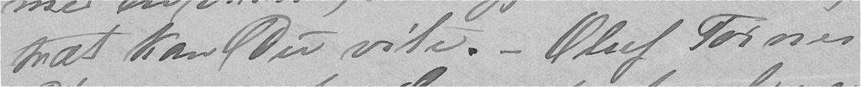træt kan Du vite. - Oluf Tornes
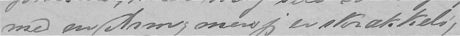med en Arm; men jeg er skrækkelig
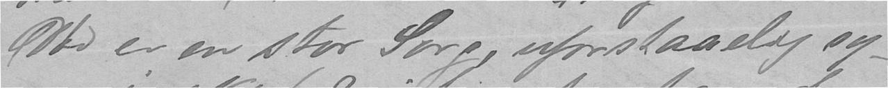Død er en stor Sorg, uforstaaelig sy-
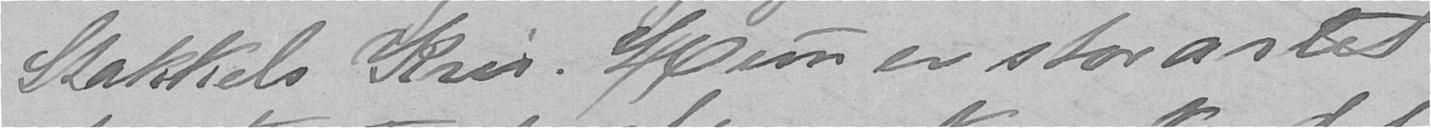Stakkels Kris. Hun er storartet.
